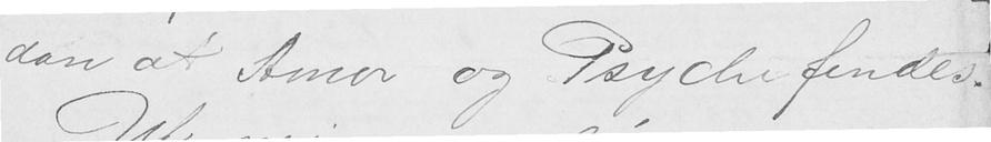dan at Amor og Psyche findes.
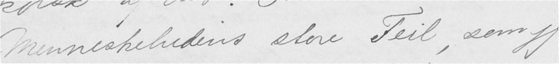Menneskehedens store Feil, som jeg
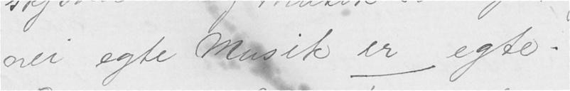nei egte Musik er egte.
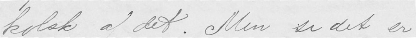kolsk af det. Men se det er
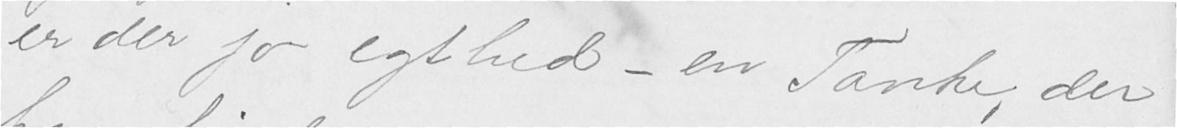er der jo egthed — en Tanke, der
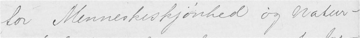for Menneskeskjønhed og Natur-
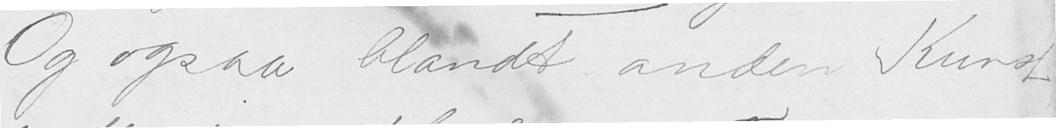Og ogsaa blandt anden Kunst
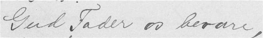Gud Fader os bevare,
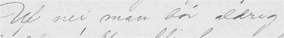Uf nei, man bør aldrig
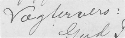Vægtervers:
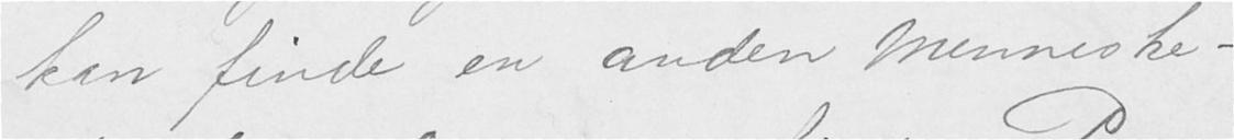kan finde en anden menneske-
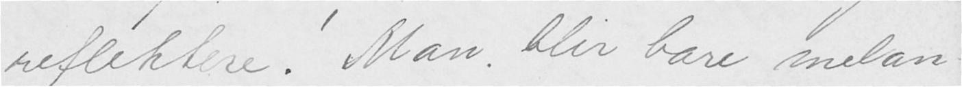reflektere! Man blir bare melan-
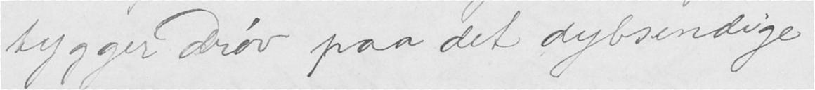tygger Drøv paa det dybsindige
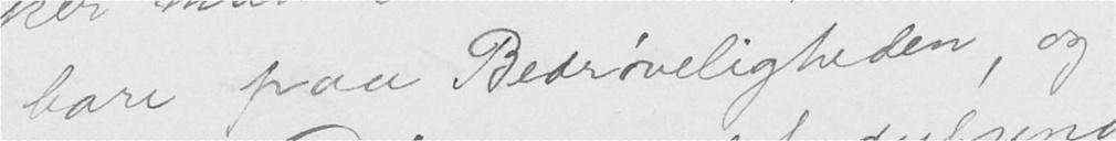bare paa Bedrøveligheden, og
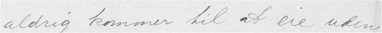aldrig kommer til at eie uden
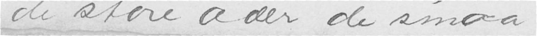de store ader de smaa —
